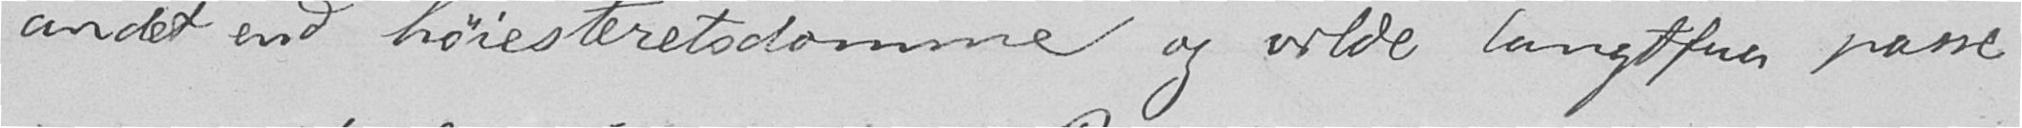andet end høiesteretsdomme og vilde langtfra passe
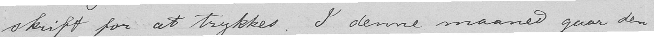skrift for at trykkes. I denne maaned gaar den
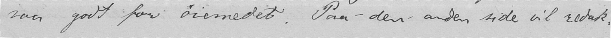saa godt for øiemedet. Paa den anden side vil redak-
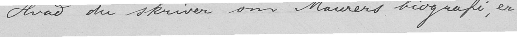Hvad du skriver om Maurers biografi, er
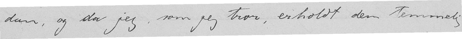dan, og da jeg, som jeg tror, erholdt den temmelig
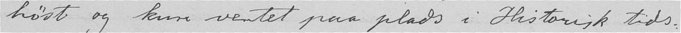høst og kun ventet paa plads i Historisk tids-
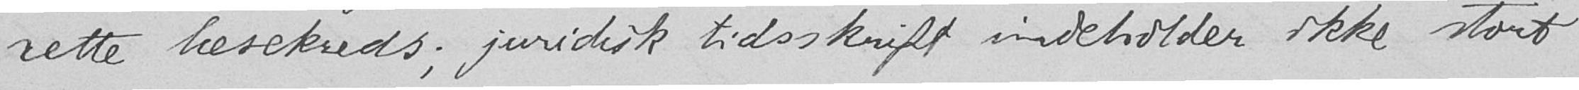rette læsekreds; juridisk tidsskrift indeholder ikke stort
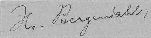Hr. Bergendahl,
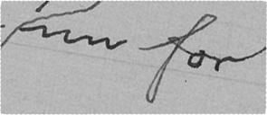nu for
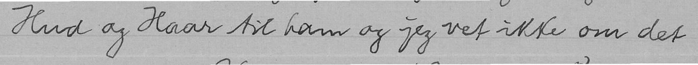Hud og Haar til ham og jeg vet ikke om det
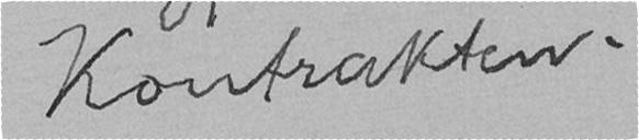Kontrakten.
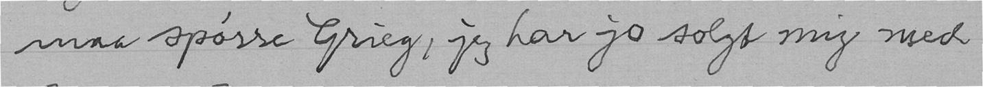maa spørre Grieg, jeg har jo solgt mig med
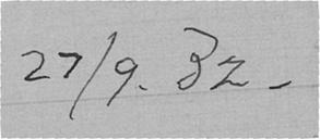27/9. 32.
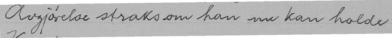Avgjørelse straks om han nu kan holde
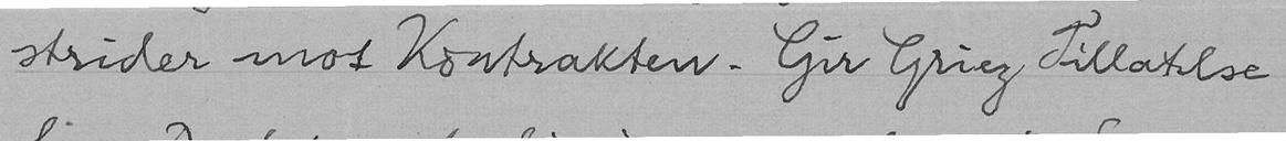strider mot Kontrakten. Gir Grieg Tillatelse
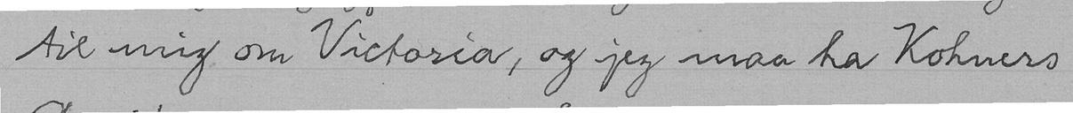til mig om Victoria, og jeg maa ha Kohners
